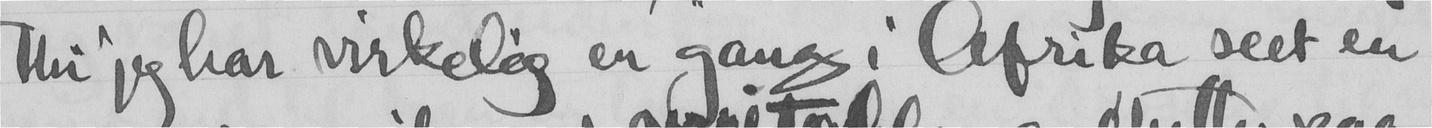thi jeg har virkelig en gang i Afrika seet en
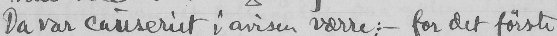Da var causeriet i avisen værre; - for det første
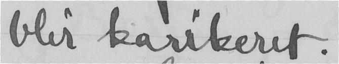blir karikeret.
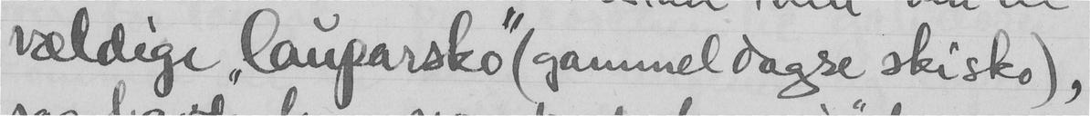vældige "lauparsko (gammeldagse skisko),
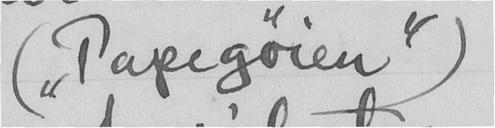("Papegøien")
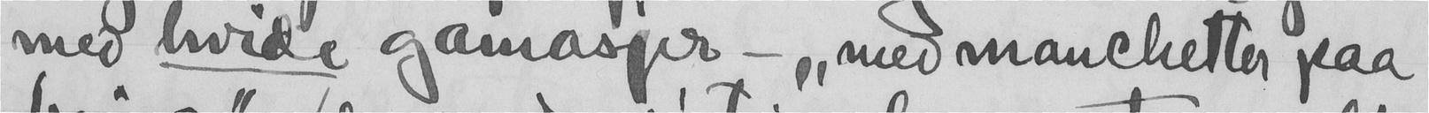med hvide gamasjer -, "med manchetter paa
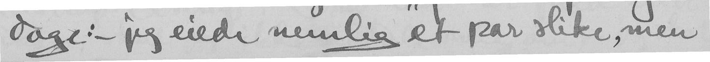dage; - jeg eiede nemlig et par slike, men
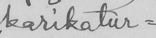karikatur-
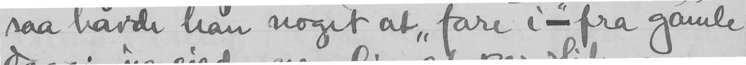saa havde han noget at "fare i" - fra gamle
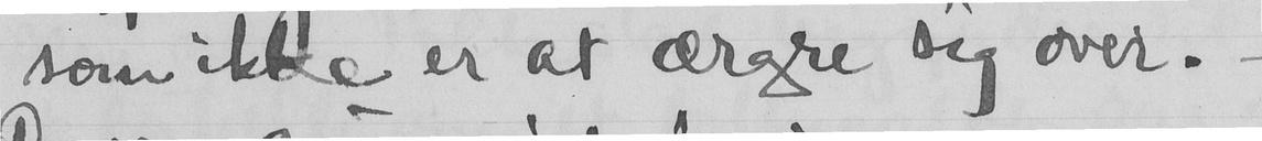som ikke er at ærgre sig over. -
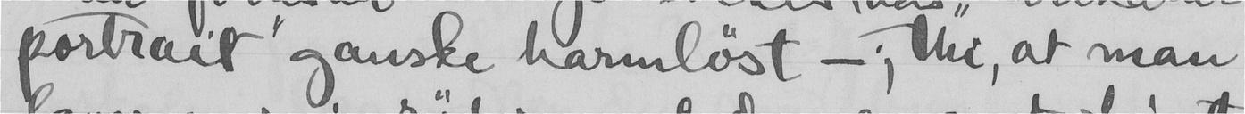portrait ganske harmløst -; thi, at man
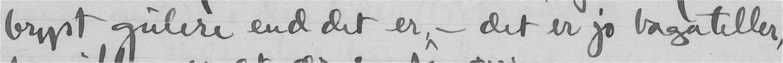bryst gulere end det er, - det er jo bagateller
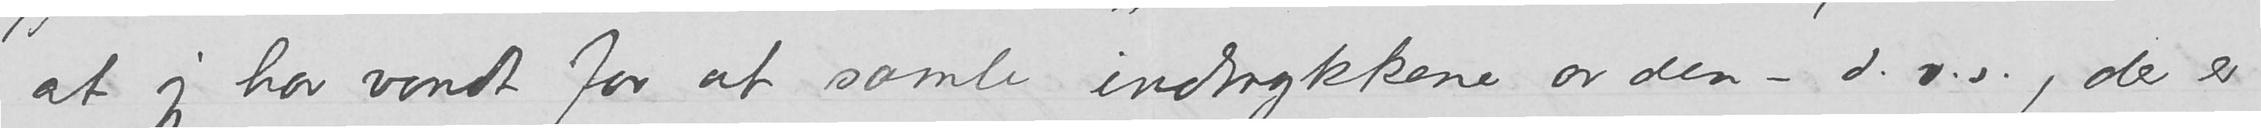at jeg har vondt for at samle indtrykkene av den – d.v.s., der er
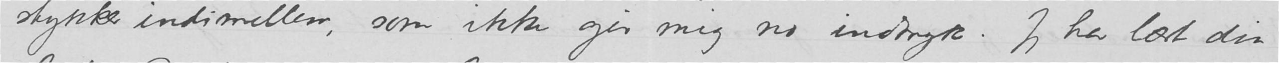stykker indimellem, som ikke gir mig no indtryk.  Jeg har læst din
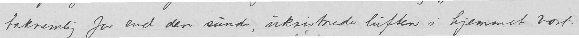taknemlig for end den sunde, ukristnede luften i hjemmet vort.
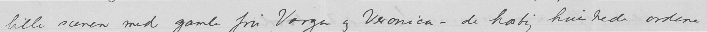lille scenen med gamle fru Vargen og Veronica – de hastig hviskede ordene
